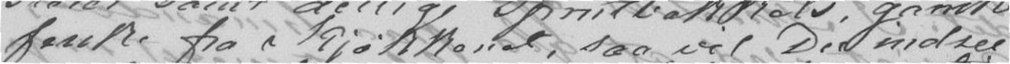ferske fra Kjøkkenet, saa vil Du indse
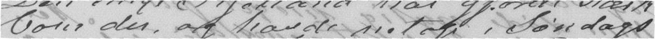Cour der, og havde netop i Søndags
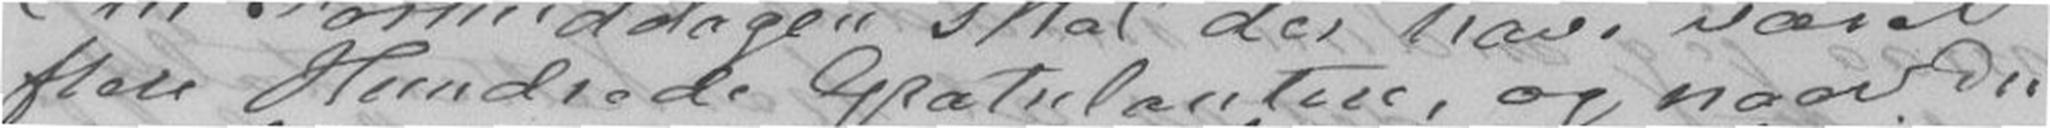flere Hundrede Gratulantere, og naar Du
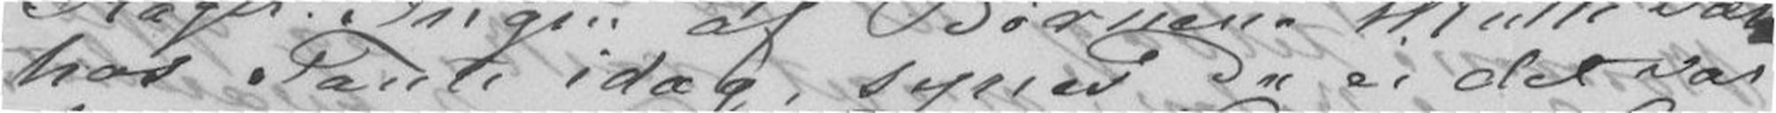hos Tante idag, synes Du ei det var
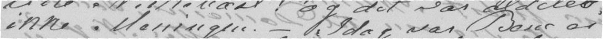ikke Meningen. - Idag var Bene ei
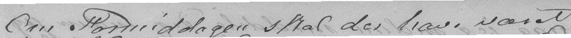Om Formiddagen skal der have været
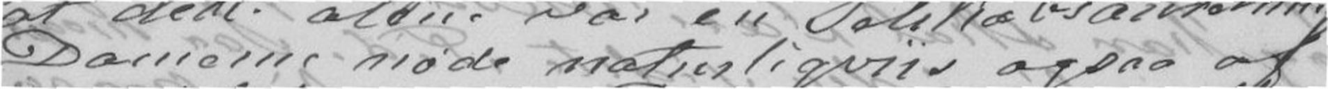Damerne nøde naturligvis ogsaa af
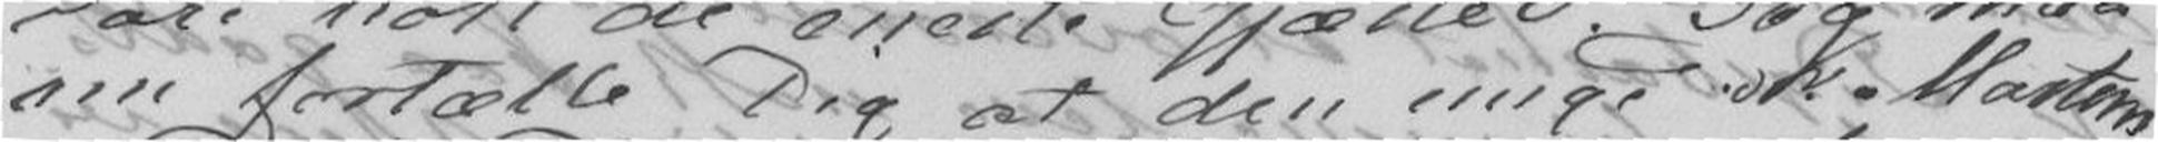nu fortælle Dig at den unge Dr Martens
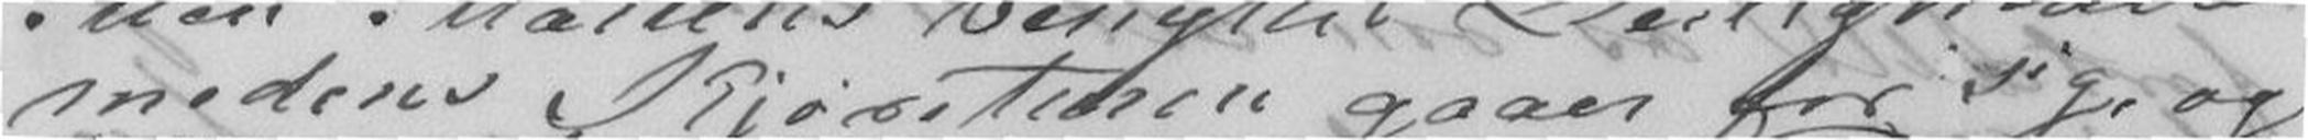medens Kjøreturen gaar for sig, og
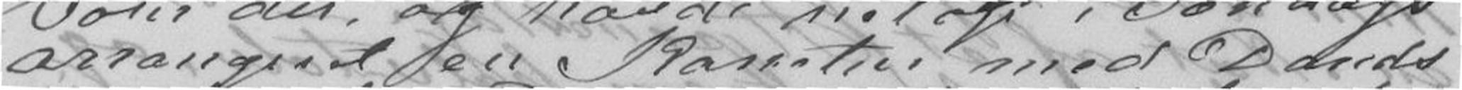arrangeret en Kanetur med Dands
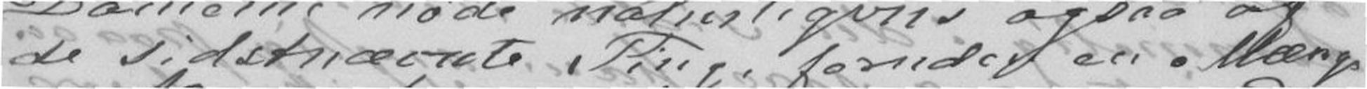de sidstnævnte Ting, foruden en Mæng-
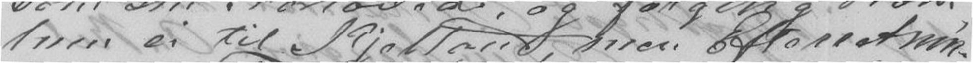hun ei til Kjelland, men Efterretnin-
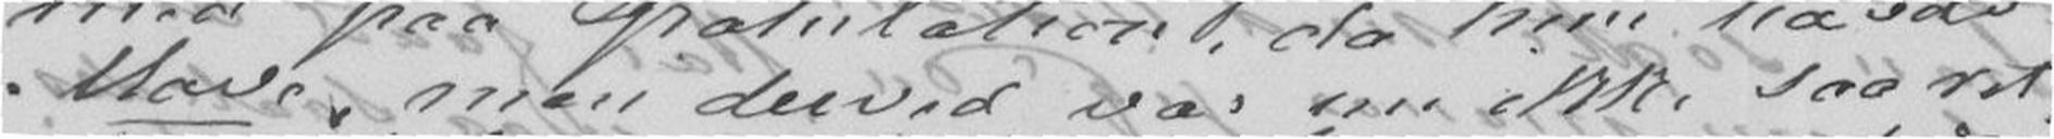Mave, men derved var nu ikke saa ret
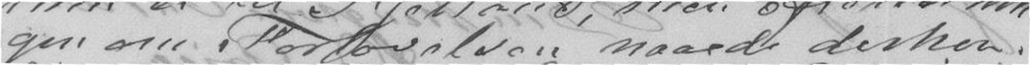gen om Forlovelsen naaede derhen.
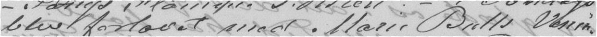blev forlovet med Marie Bulls Venin-
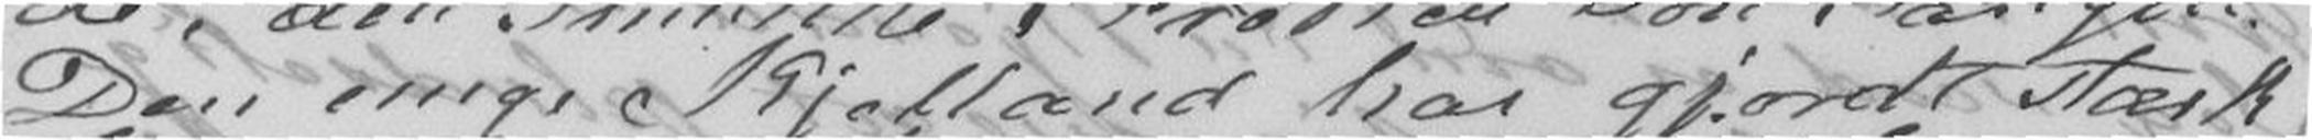Den unge Kjelland har gjort stærk
